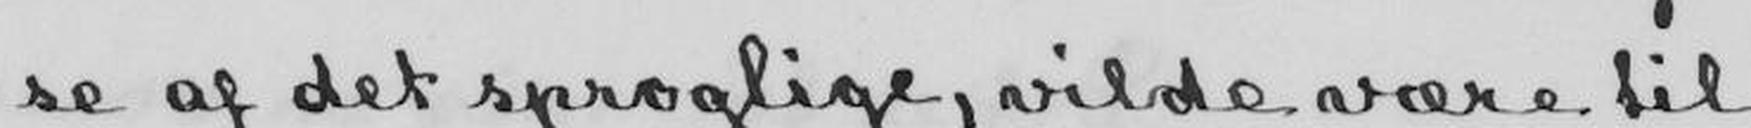se af det sproglige, vilde være til
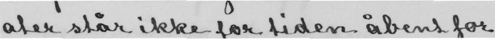ater står ikke for tiden åbent for
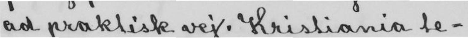ad praktisk vej. Kristiania te-
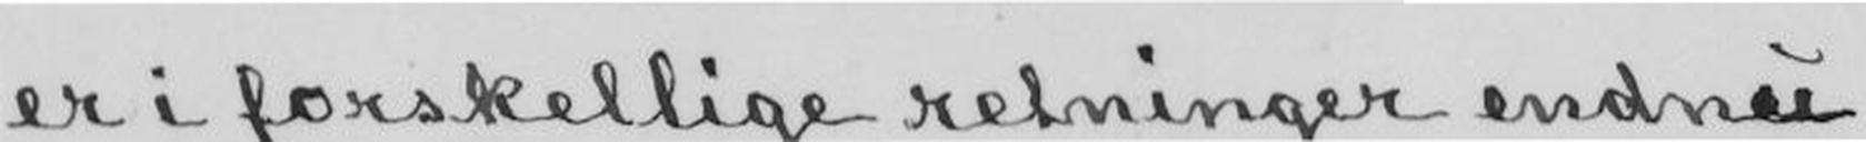er i forskellige retninger endnu
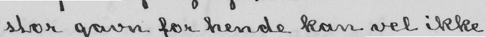stor gavn for hende kan vel ikke
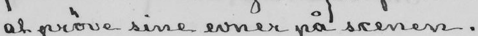at prøve sine evner på scenen.
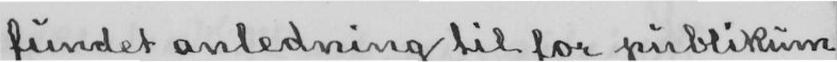fundet anledning til for publikum
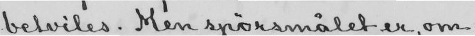betviles. Men spørgsmålet er, om
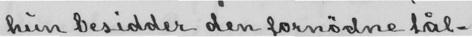hun besidder den fornødne tål-
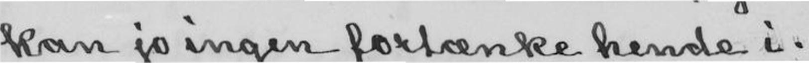kan jo ingen fortænke hende i.
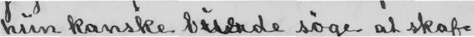hun kanske burde søge at skaf-
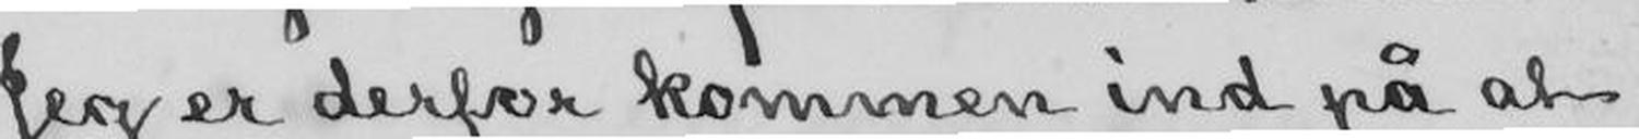Jeg er derfor kommen ind på at
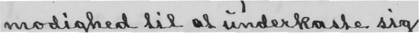modighed til at underkaste sig
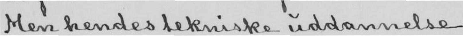Men hendes tekniske uddannelse
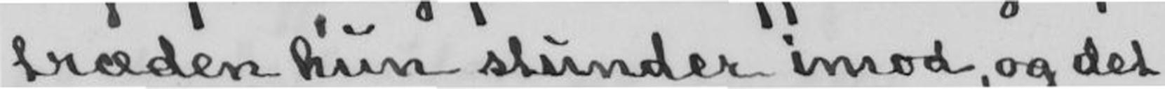træden hun stunder imod, og det
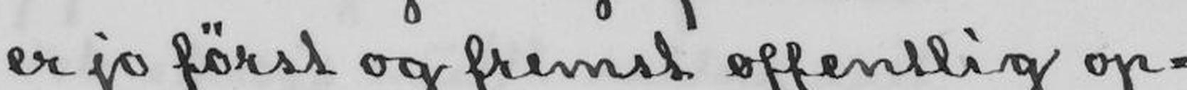er jo først og fremst offentlig op-
